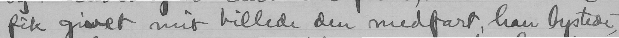fik givet mit billede den medfart, han lystede,
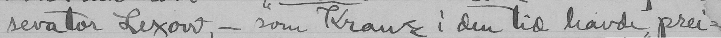sevator Lexon - som Kranz i den tid havde "prei-
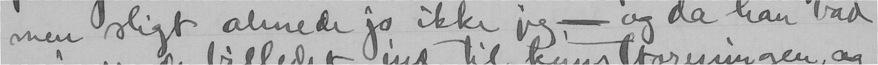men sligt ahnede jo ikke jeg - og da han bad
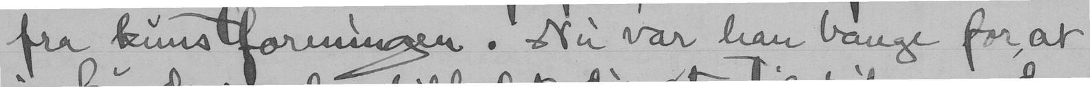fra kunstforeningen. Nu var han bange for, at
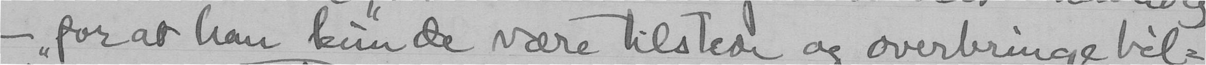-"for at han kunde være tilstede og overbringe bil-
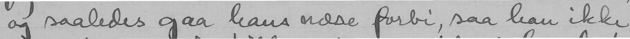og saaledes gaa hans næse forbi, saa han ikke
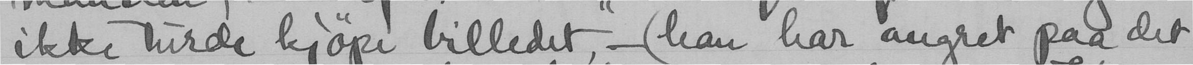ikke turde kjøpe billedet", - (han har angret paa det
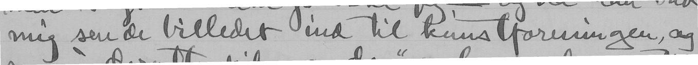mig sende billedet ind til kunstforeningen, og
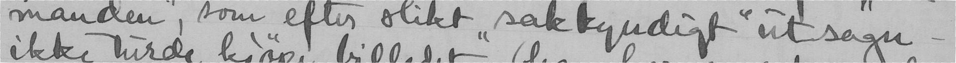manden", som efter slikt "sakkyndigt" utsagn -
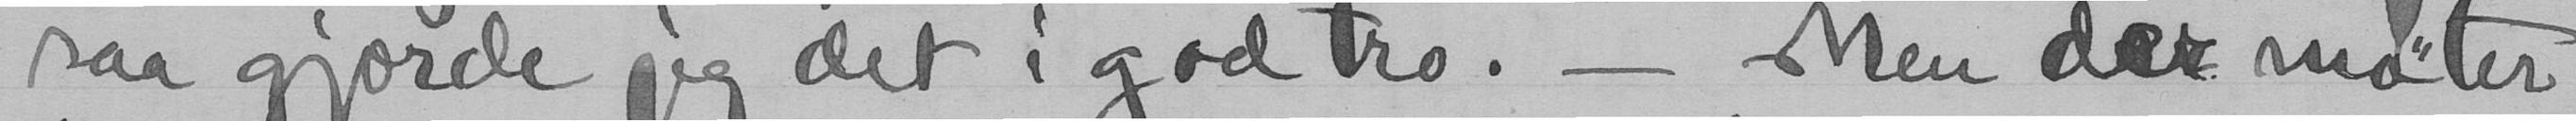saa gjorde jeg det i god tro. - Men der møter
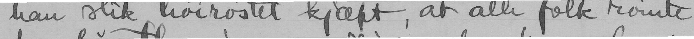han slik høirøstet kjæft, at alle folk rømte
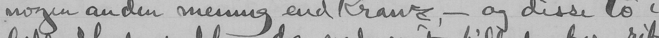nogen anden mening end Kranz, - og disse to i
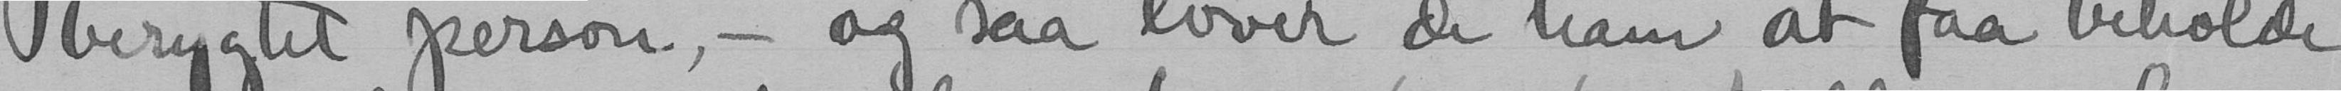berygtet person, - og saa lover de ham at faa beholde
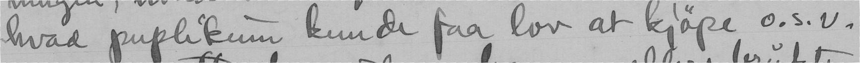hvad puplikum kunde faa lov at kjøpe o.s.v.
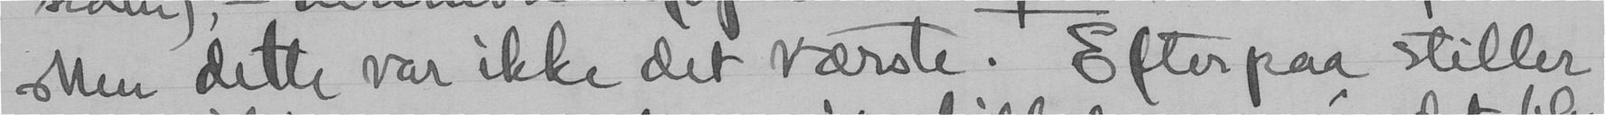Men dette var ikke det værste. Efterpaa stiller
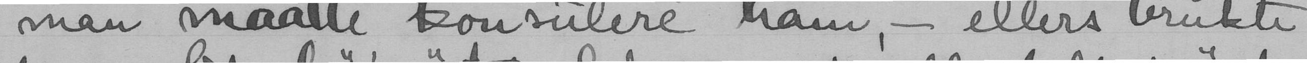man maatte konsulere ham, - ellers brukte
